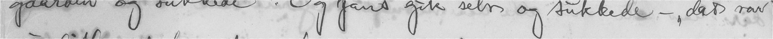gaarden og sukkede. Og Jans gik selv og sukkede - "dat var
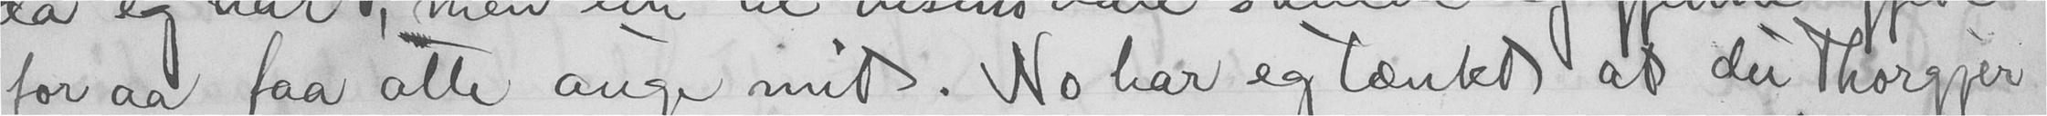for aa faa atte auge mit. No har eg tænkt at du Thorgjer
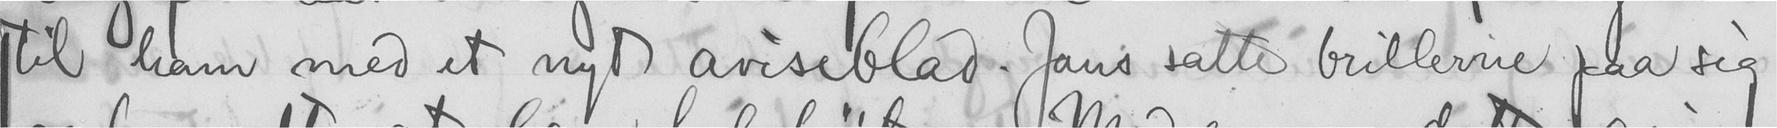til ham med et nyt aviseblad. Jans satte brillerne paa sig
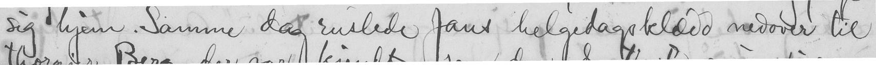sig hjem. Samme dag ruslede Jans helgedagsklædd nedover til
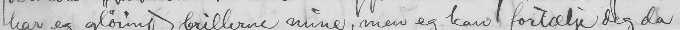har eg gløimt, brillerne mine, men eg kan fortælje deg da
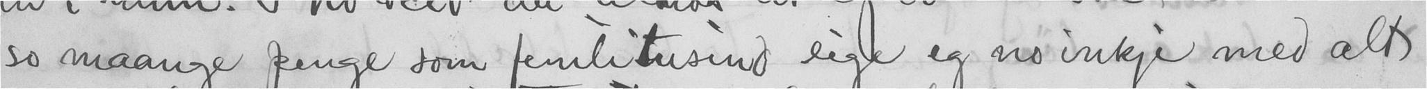so maange penge som femtitusind lige eg no inkje med alt
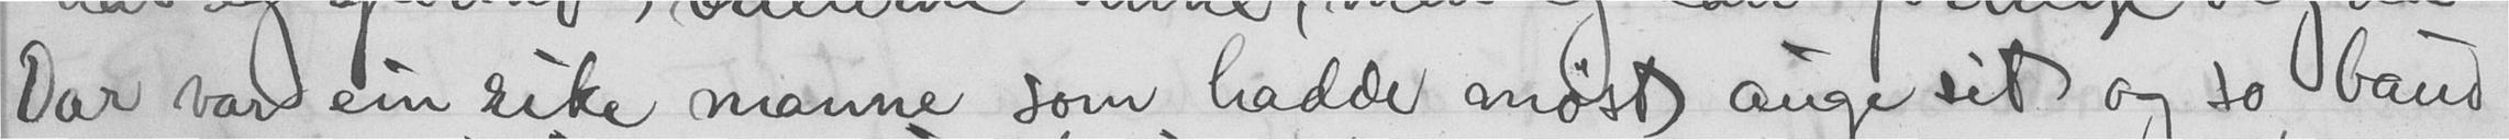Dar var ein rike manne som hadde møst auge sit og so baud
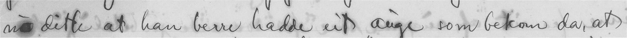nu ditte at han berre hadde eit auge som bekom da, at
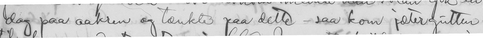dag paa aakren og tænkte paa dette - saa kom jætergutten
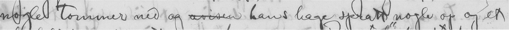nogle tommer ned og  hans hage spratt nogle op og et
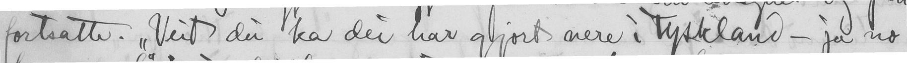fortsatte. "Veit du ka dei har gjort nere i Tyskland - ja no
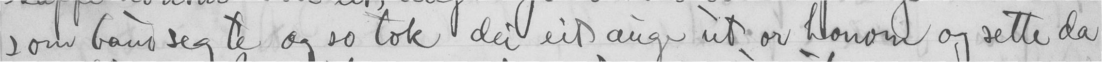som baud sig te og so tok dei eit auge ut or honom og sette da
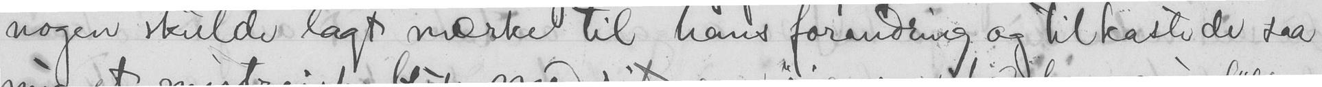nogen skulde lagt mærke til hans forandring og tilkaste de saa
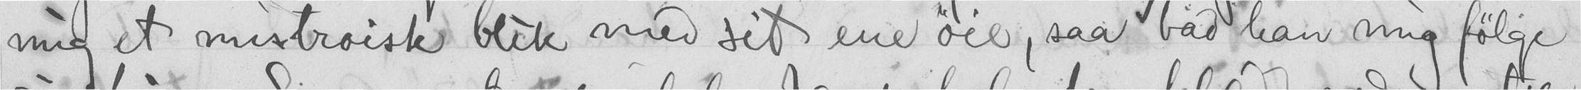mig et mistroisk blik med sit ene øie, saa bad han mig følge
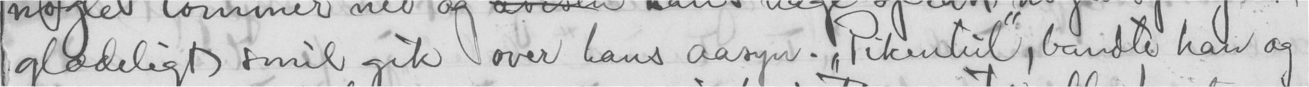glædeligt smil gik over hans aasyn. "Pikentul", bandte han og
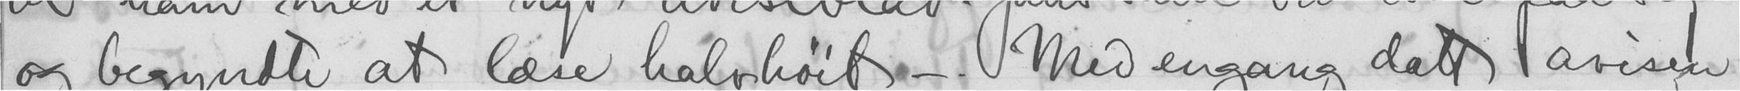og begyndte at læse halvhøit. - Med engang datt avisen
